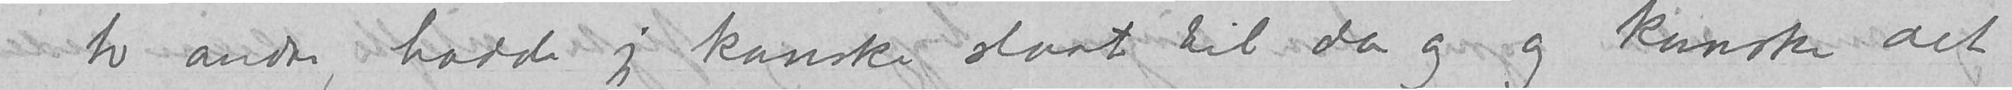to andre, hadde jeg kanske slaat til da og og kanske det
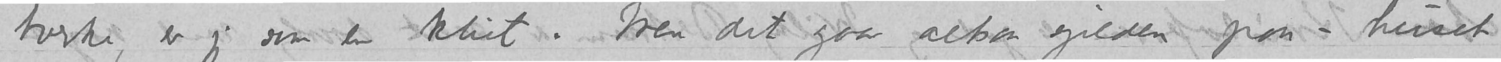tverke, er jeg som en klut. Men det gaar altsaa gilden/spilden/gulde paa – huset
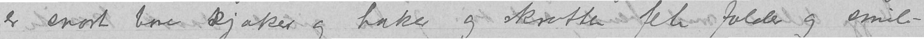er snart bare kjaker og haker og skrotten fete folder og smile-
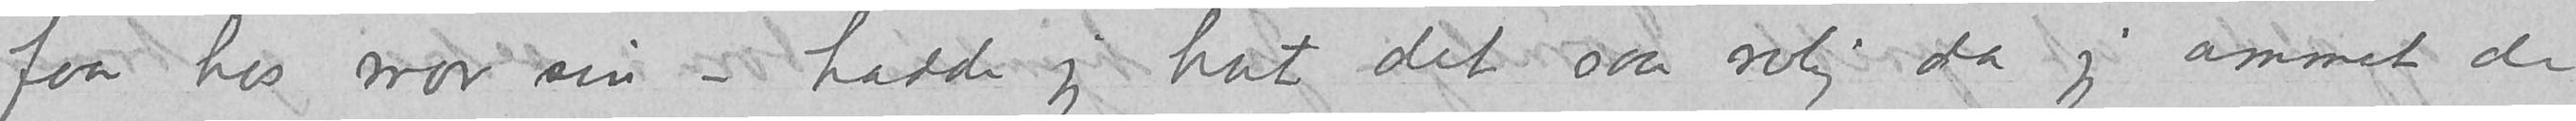faar hos mor sin – hadde jeg hat det saa rolig da jeg ammet de
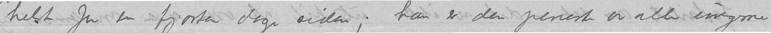helst for en fjorten dager siden; han er den peneste av alle ungerne
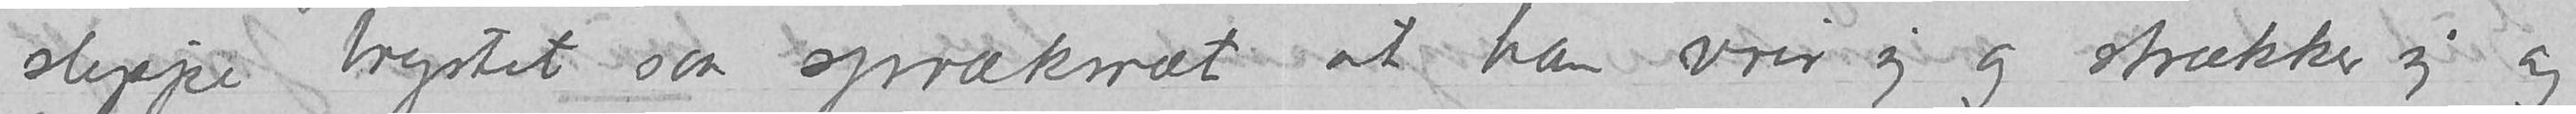slippe brystet saa sprækmæt at han vrir sig og strækker sig og
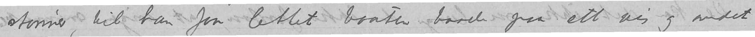stønner, til han faar lettet baaten baade paa ett vis og andet
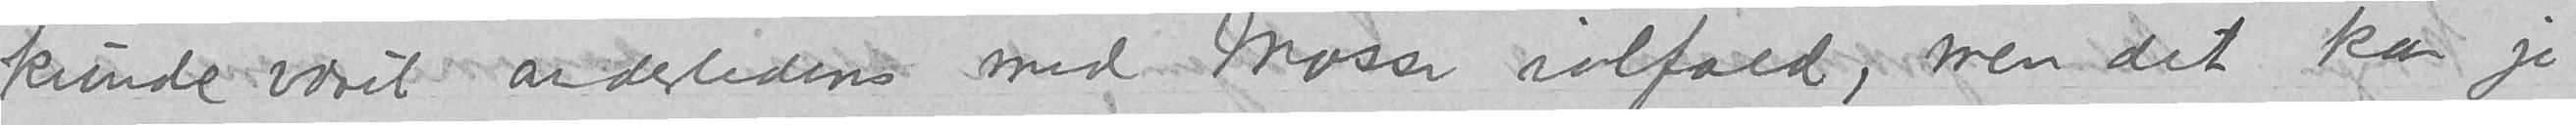kunde været anderledens med Mosse ialfald, men det kan jo
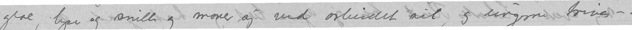glae, lyse og snille og morer sig ved arbeidet sit, og ungerne trives –.
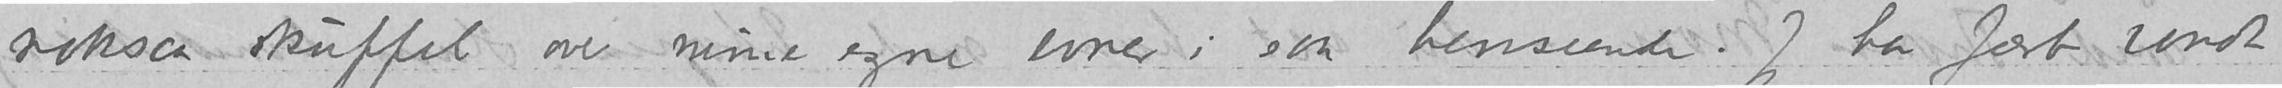noksaa skuffet over mine egne evner i saa henseende. Jeg har færb vondt
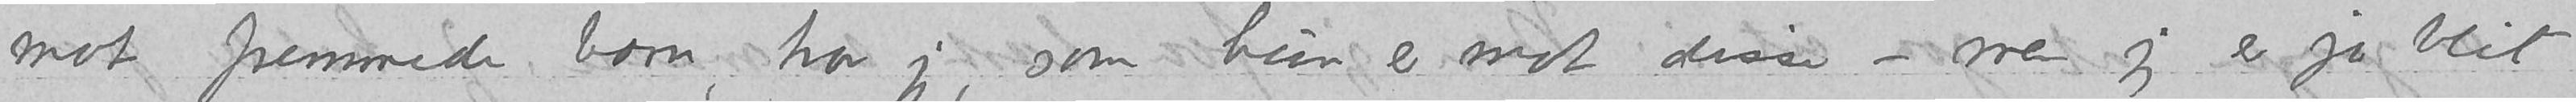mot fremmede barn, tror jeg, som hun er mot disse – men jeg er jo blit
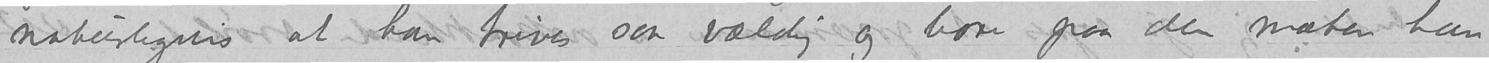naturligvis at han trives saa vældig og bare paa den maten han
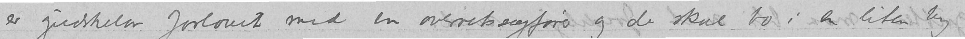er gudskelov forlovet med en overretssagsfører og de skal bo i en liten by
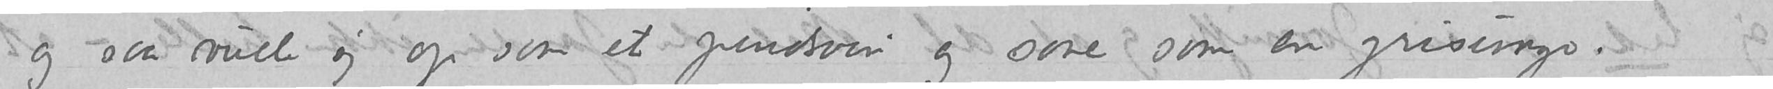og saa ruller sig op som et pindsvin og sove som en griseunge.
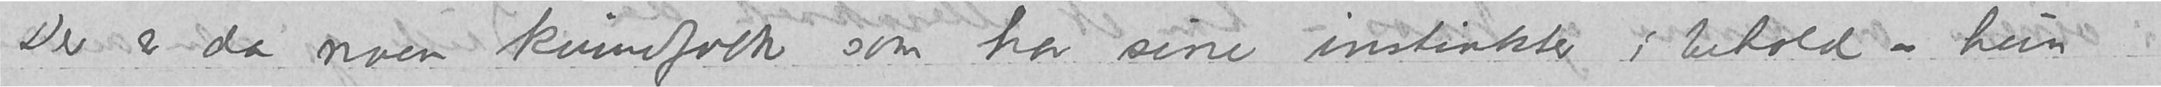Der er da noen kvindfolk som har sine instinkter i behold – hun
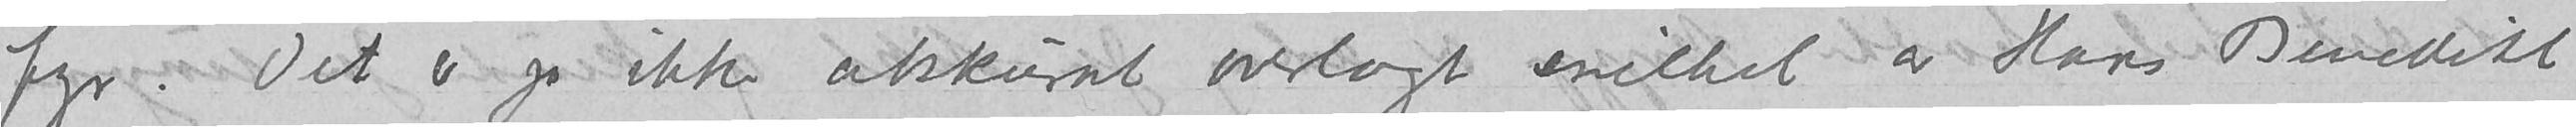fyr. Det er jo ikke akkurat overlagt snilhet av Hans Benedikt
